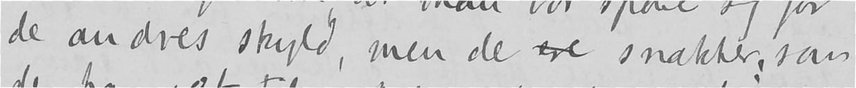de andres skyld, men de ere snakker, som
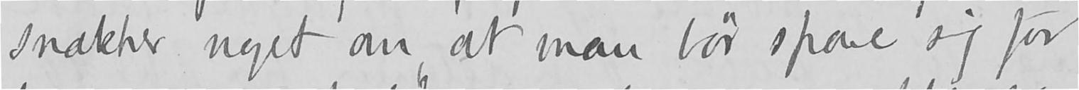snakker noget om, at man bør spare sig for
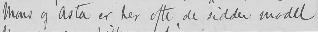Mons og Asta er her ofte, de sidder model
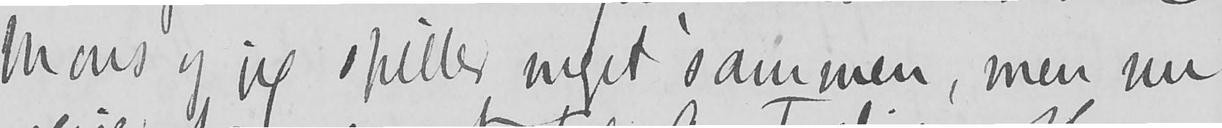Mons og jeg spiller meget sammen, men nu
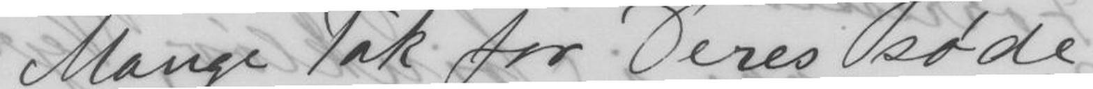Mange Tak for Deres søde
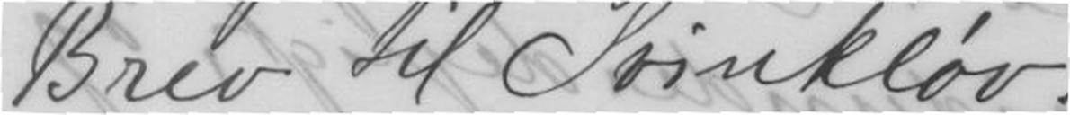Brev til Svinkløv.
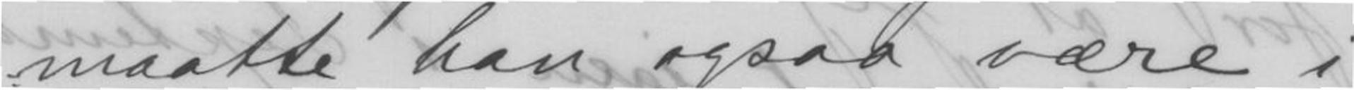maatte han ogsaa være i
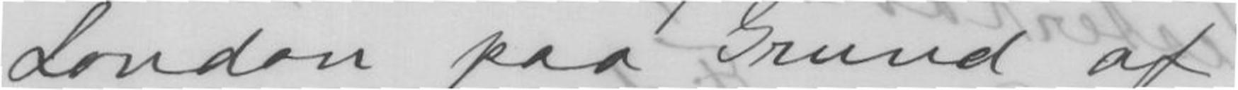London paa Grund af
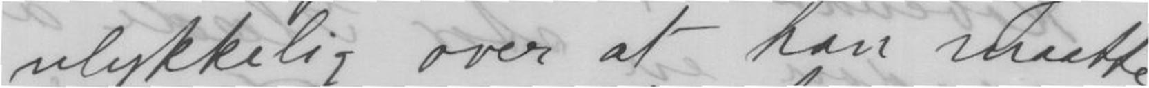ulykkelig over at han maatte
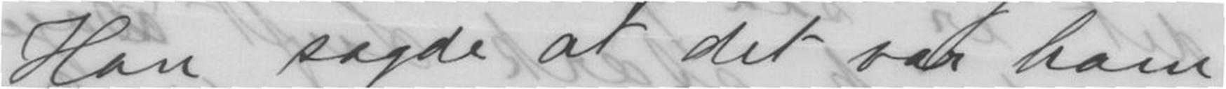Hans sagde at det var ham
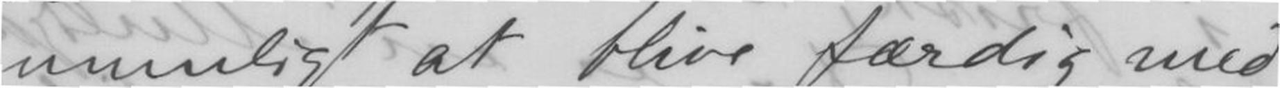muligt at blive færdig med
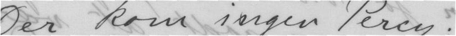Der kom ingen Percy.
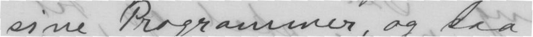sine Programmer, og saa
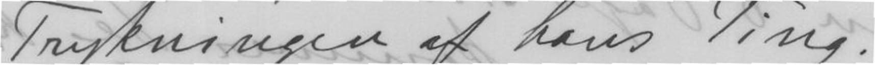Trykningen af hans Ting.
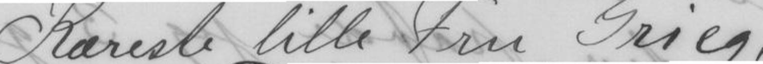Kæreste lille Fru Grieg,
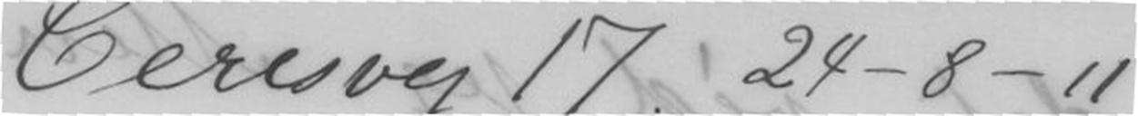Ceresvej 17. 24-8-11
